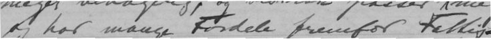og har mange Fordele fremfor Fattig
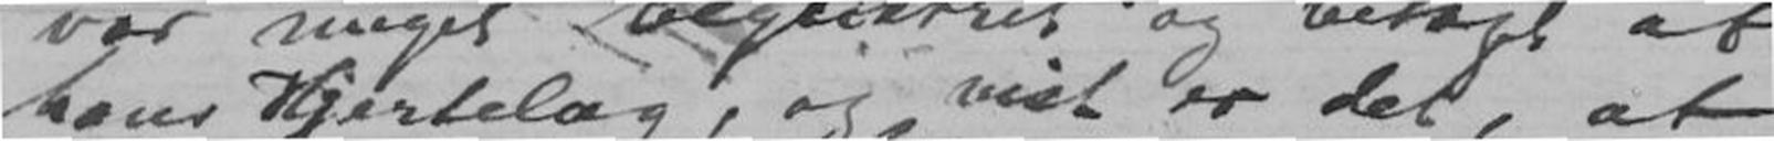hans Hjertelag, og vist er det, at
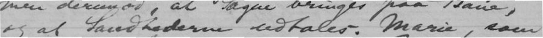og at Sandhederne udtales. Marie, som
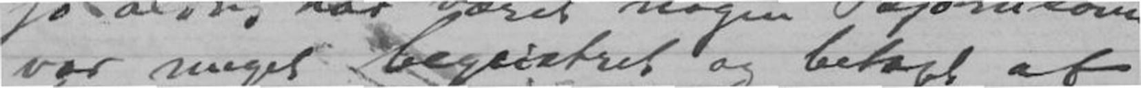var meget begeistret og betaget af
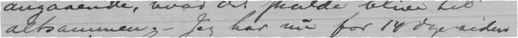altsammen. - Jeg har nu for 14 Dage siden
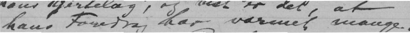hans Foredrag har varmet mange.
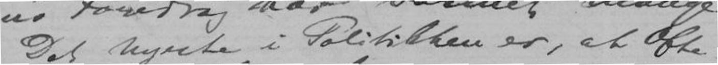- Det nyeste i Politikken er, at Ofte-
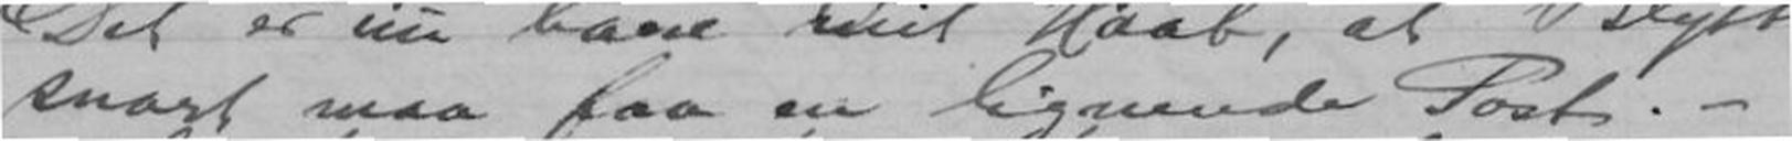snart maa faa en lignende Post. -
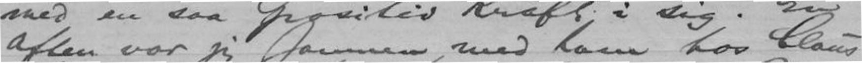Aften var jeg sammen med ham hos Claus
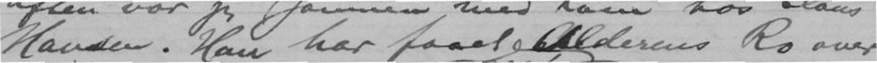Hansen. Han har faaet Alderens Ro over
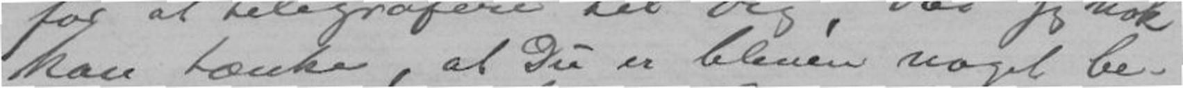kan tænke, at Du er bleven noget be-
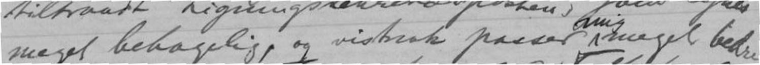meget behagelig, og vistnok passer meget bedre
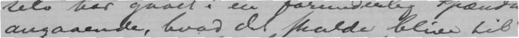angaaende, hvad det skulde blive til
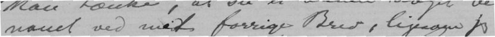nauet ved mit forrige Brev, ligesaa jeg
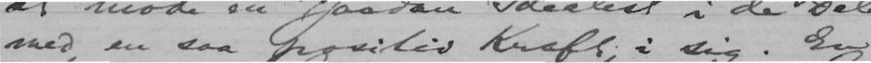med en saa positiv Kraft i sig. En
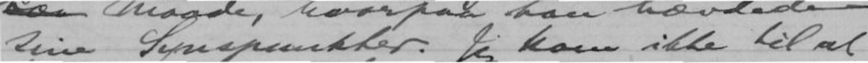sine Synspunkter. Jeg kom ikke til at
